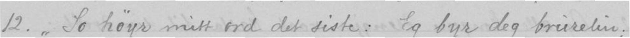12. So høyr mitt ord det siste: Eg byr deg brurelin;
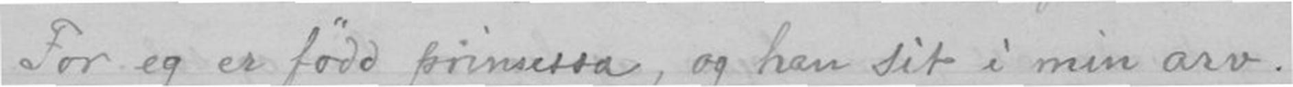For eg er fødd prinsessa, og han sit i min arv.
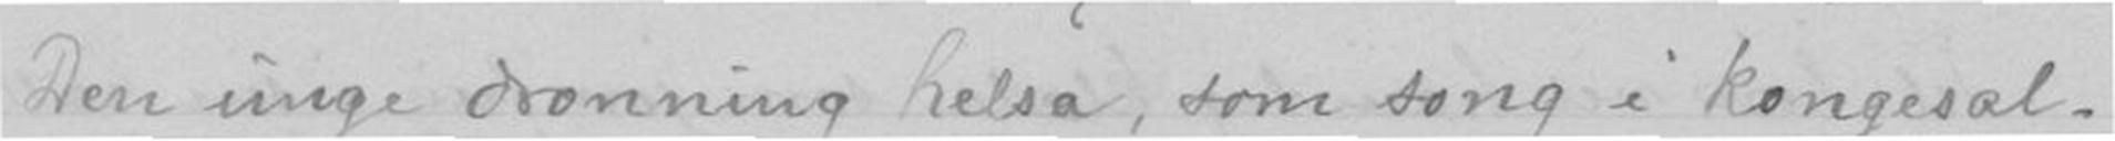Den unga dronning helsa, som song i kongesal.
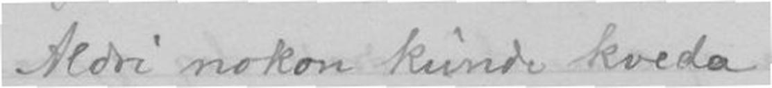Aldri nokon kunde kveda
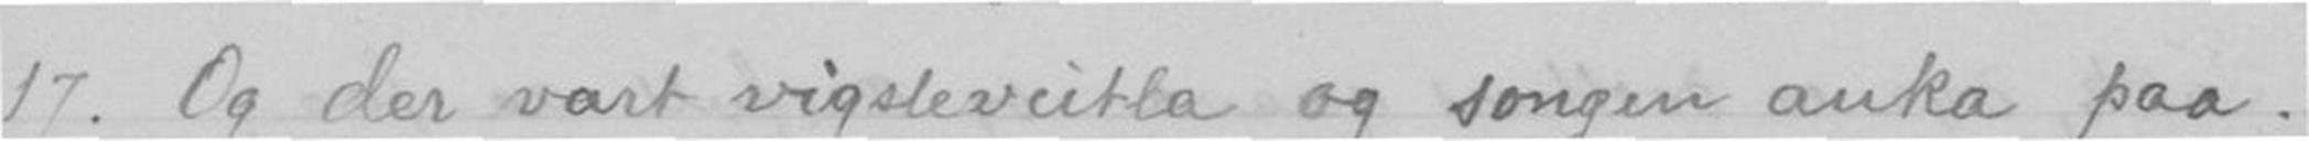17. Og der vart vigsleveitla og songen auka paa.
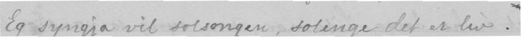Eg syngja vil solsangen, solenge det er liv.
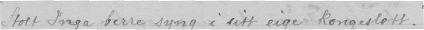Stolt Inga berre syng i sitt eiga kongeslott.
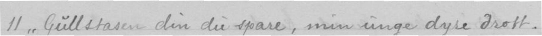11. Gullstasen din du spare, min unge dyre drott.
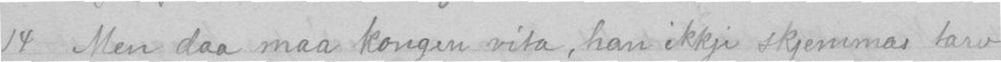14. Men daa maa kongen vita, han ikkje skjemmas tarv,
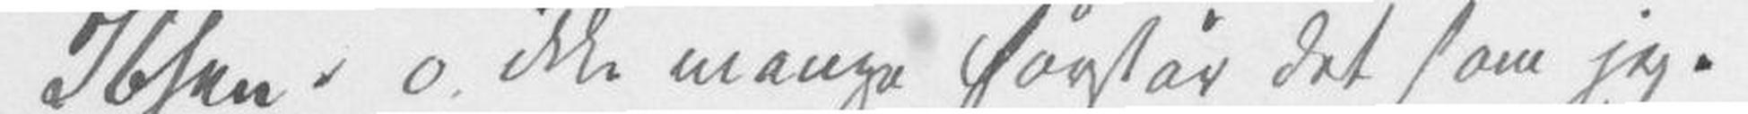Ibsen, o ikke mange forstår det som jeg.
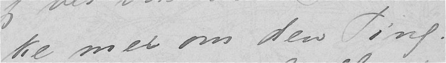ke mer om den Ting.
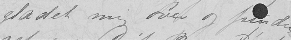glædet nu over og funde-
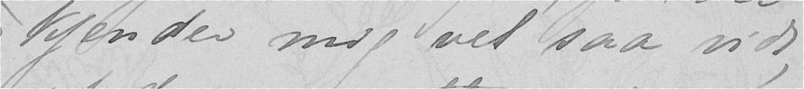kjender mig vel saa vidt,
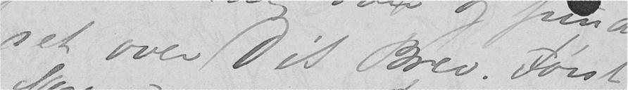ret over Dit Brev. Først
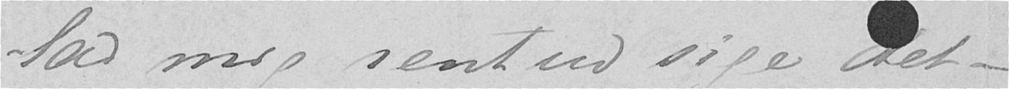lad mig rent ud sige det -
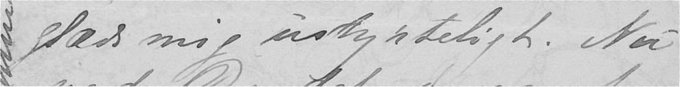glæde mig ustyrteligt. Nu
